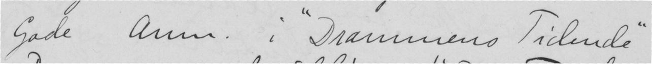Gode Anm. i "Drammens Tidende"
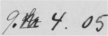9. 4.05
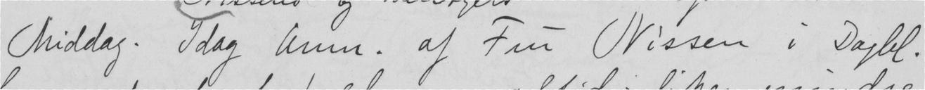Middag. Idag Anm. af Fru Nissen i Dagbl.
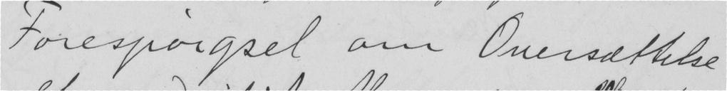Forespørgsel om Oversættelse
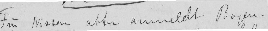Fru Nissen atter anmeldt Bogen.
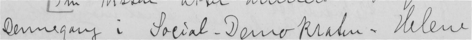Dennegang i Social-Demokraten. Helene
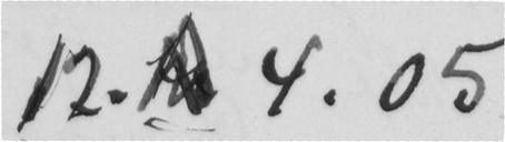12. 4.05
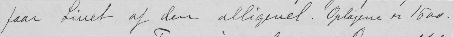faar Livet af den alligevel. Oplagene er 1500.
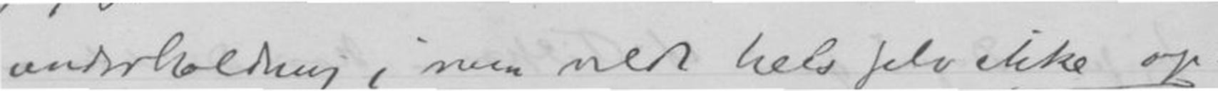underholdning, men vilde hels selv ikke op-
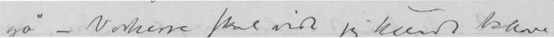gå - Vorherre skal vide jeg kunde beskrive
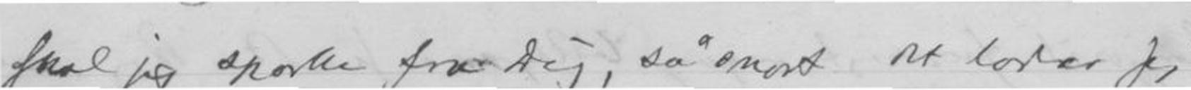jeg sparke for Dig, så snart det lader sig
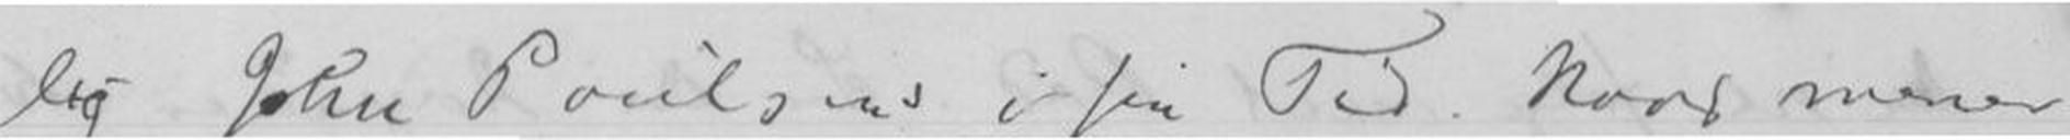lig John Poulsens i sin Tid. Hvad mener
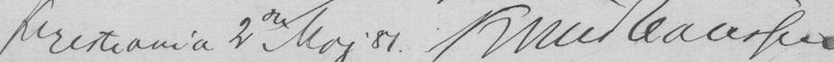Kristiania 2dre Maj 81Knud Claussen
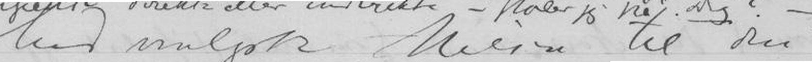Med venligst Hilsen til Din
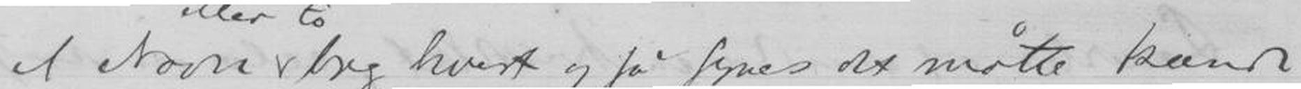et større breg hvert og så synes det måtte kunde
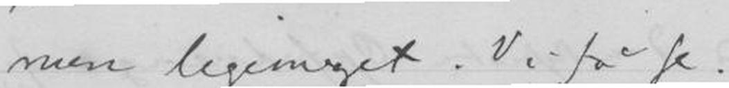men ligemeget. Vi får se.
